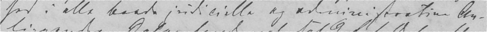hed i alle baade judicielle og administrative An-
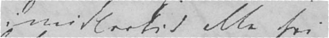imidlertid alle fri
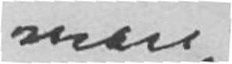man
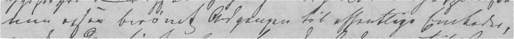men ogsaa berøvet Adgangen til offentlige Embeder,
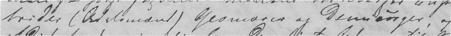briser (Adelsmænd) Gesmares og Demsorger, og
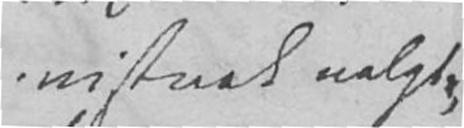vistnok valgte,
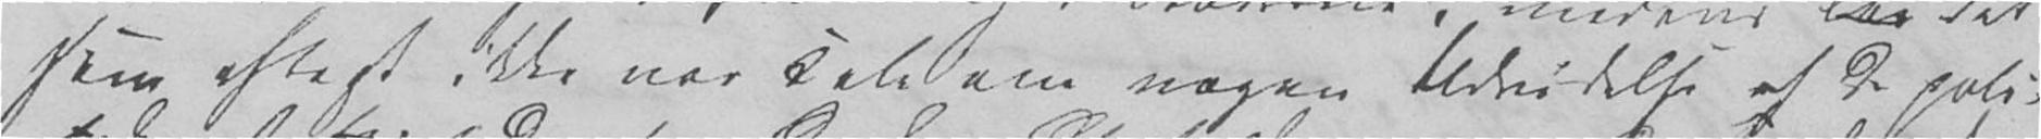som oftest ikke var Tale om nogen Udvidelse af de poli-
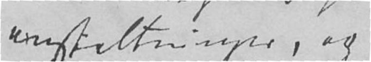anstaltninger, og
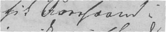fik Overhaand i
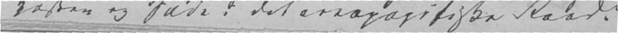Kæsten og Sæde i det areopagitiske Raad.
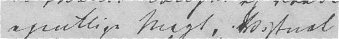egentlige Magt. Vistnok
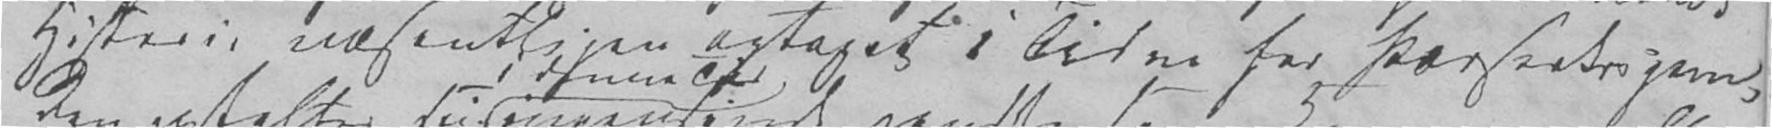Historie væstentligen optaget i Tiden for Perserkrigen,
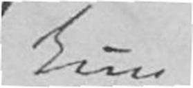kun
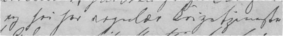og fri for regulær Krigstjeneste
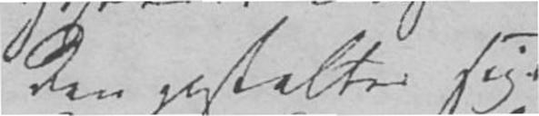den gestalter sig
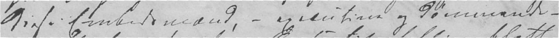disse Embedsmænd, – executive og dømmende–
